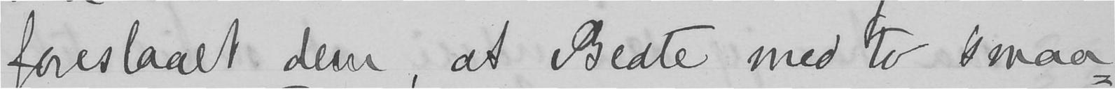foreslaaet dem, at Beate med to smaa-
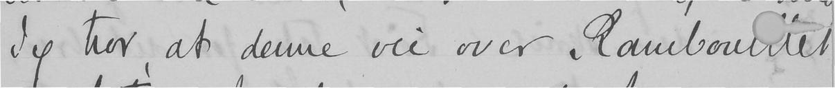Jeg tror, at denne vei over Rambouillet
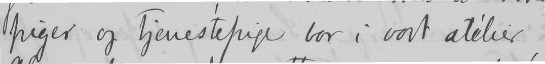piger og tjenestepige bor i vort atélier,
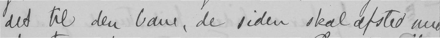det til den bane, de siden skal afsted med
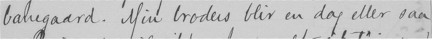banegaard. Min broders blir en dag eller saa
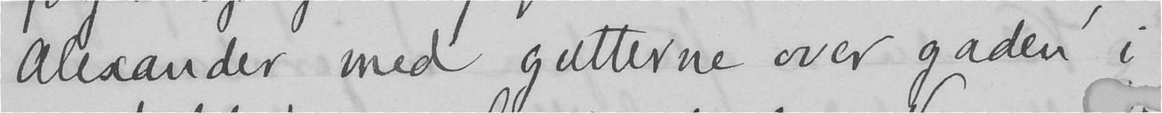Alexander med gutterne over gaden i
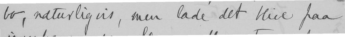bo, naturligvis, men lade det blive paa
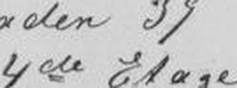4de Etage
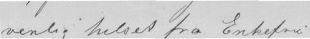venlig hilset fra Enkefru
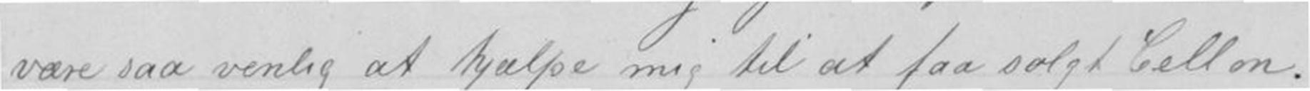være saa venlig at hjælpe mig til at faa solgt Cellon.
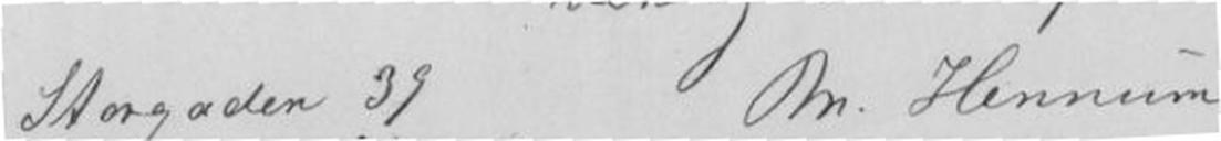Storgaden 39M. Hennum.
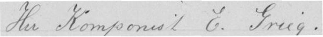Hrr Komponist E. Grieg.
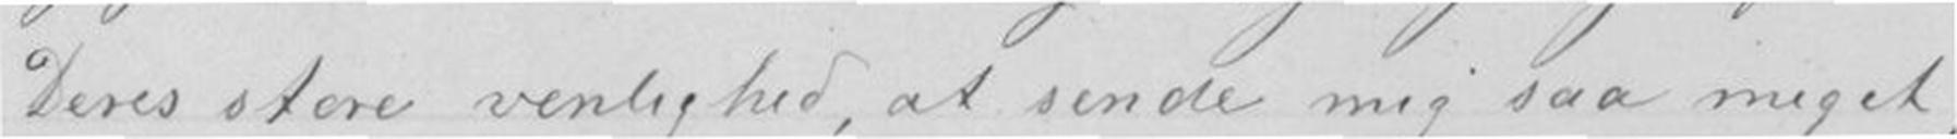Deres store venlighed, at sende mig saa meget,
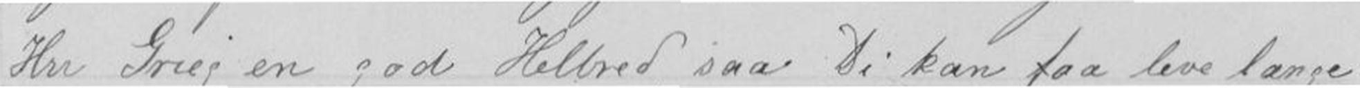Hrr Grieg, en god Helbred, saa Di kan faa leve længe
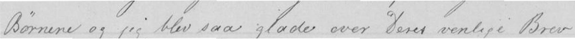Børnene og jeg, blev saa glade over Deres venlige Brev
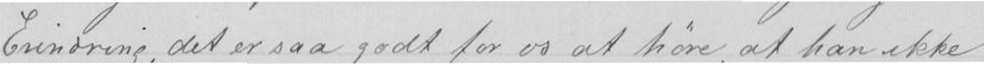Erindring, det er saa godt for os at høre, at han ikke
